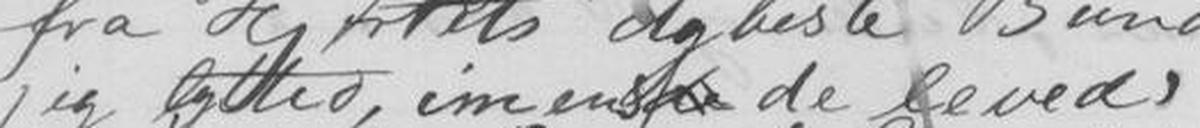jeg lytted, imens de leved,
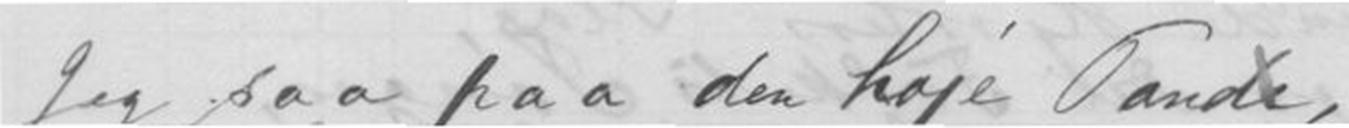Jeg saa paa den høje Pande,
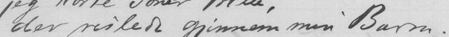der vislede gjennem min Barm.
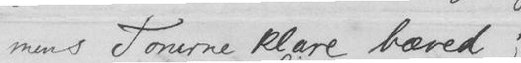mens Tonerne klare bæred;
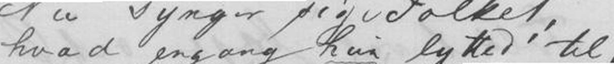hvad engang hun lytted til,
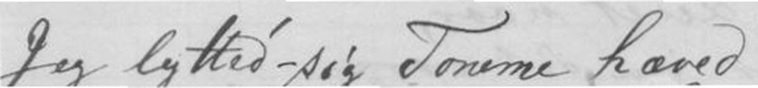Jeg lytted-søg Tonerne hæved
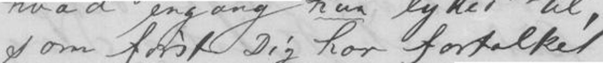som først Dig har fortolket
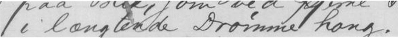i længtende Drømme hang.
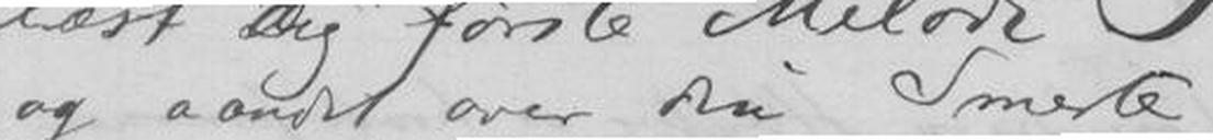og aandet over din Smerte
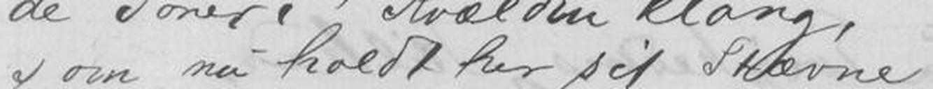som nu holdt her sit stævne
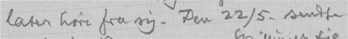later høre fra sig. Den 22/5. sendte
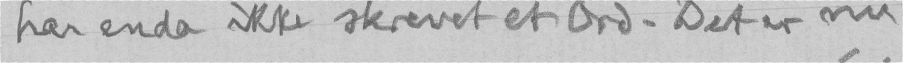har enda ikke skrevet et Ord. Det er nu
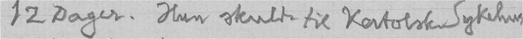12 Dager. Hun skulde til Katolske Sykehus
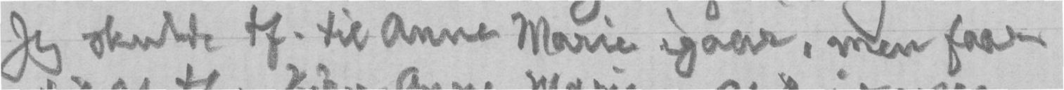Jeg skulde tf. til Anne Marie igaar, men faar
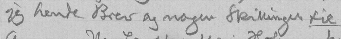jeg hende Brev og nogen Skillinger til
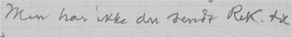Men har ikke du sendt Rek. til
